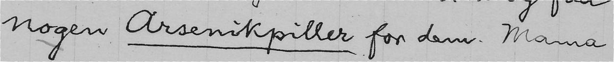nogen Arsenikpiller for dem. Mama
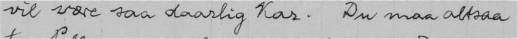vil være saa daarlig Kar. Du maa altsaa
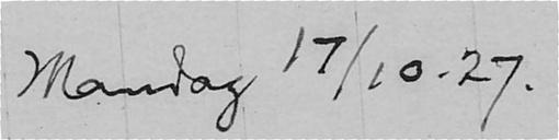Mandag 17/10. 27.
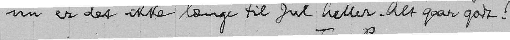nu er det ikke længe til Jul heller. Alt gaar godt!
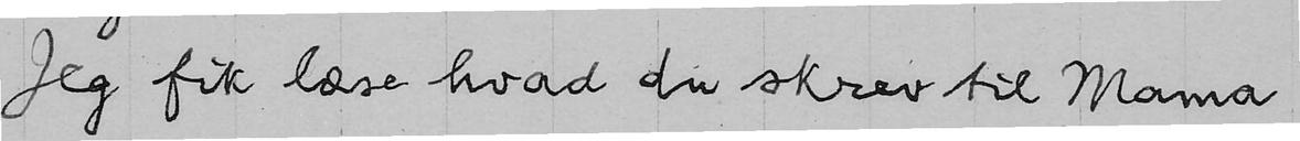Jeg fik læse hvad du skrev til Mama
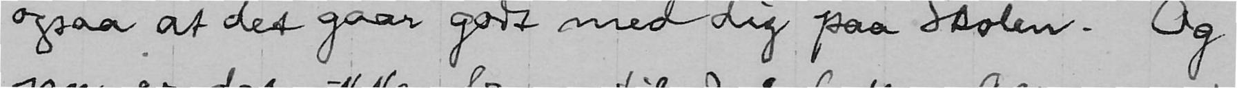ogsaa at det gaar godt med dig paa Skolen. Og
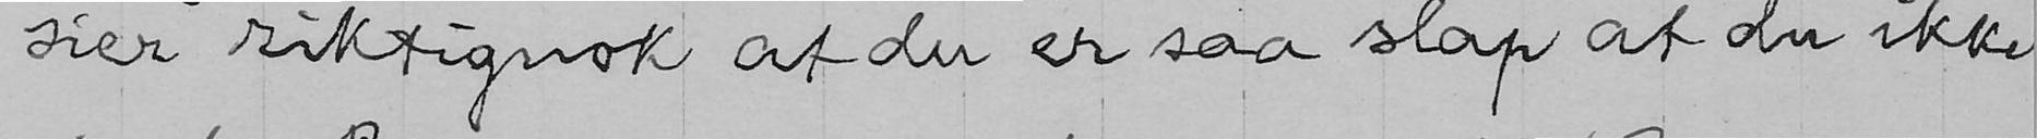sier riktignok at du er saa slap at du ikke
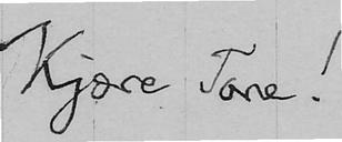Kjære Tore!
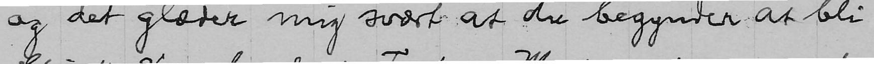og det glæder mig svært at du begynder at bli
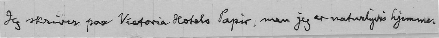Jeg skriver paa Victoria Hotels Papir, men jeg er naturligvis hjemme.
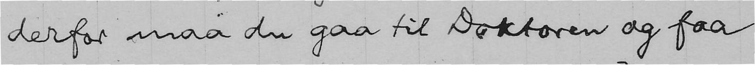derfor maa du gaa til Doktoren og faa
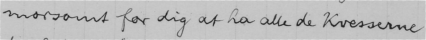morsomt for dig at ha alle de Kvesserne
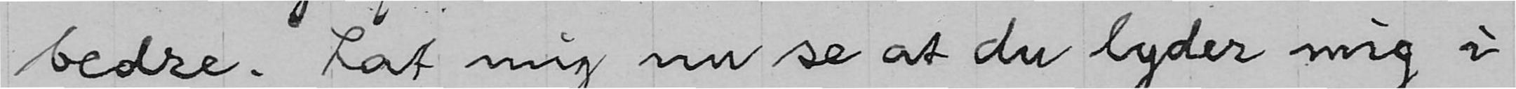bedre. Lat mig nu se at du lyder mig i
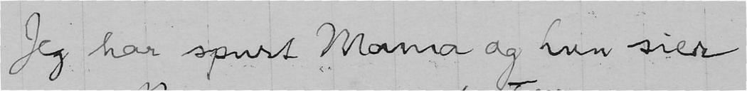Jeg har spurt Mama og hun sier
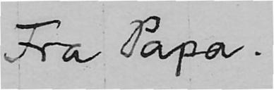Fra Papa.
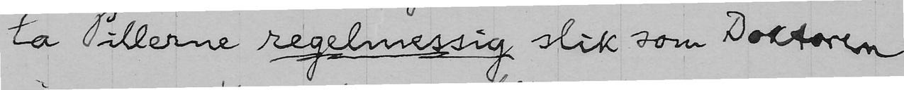ta Pillerne regelmessig slik som Doktoren
